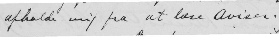afholde mig fra at læse aviser.
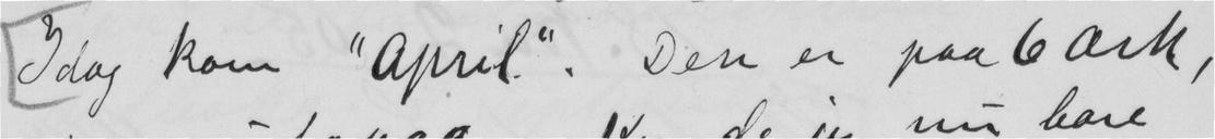Idag kom "April". Den er paa 6 Ark,
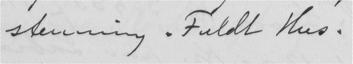stemning. Fuldt Hus.
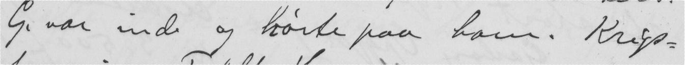G. var inde og hørte paa ham. Krigs-
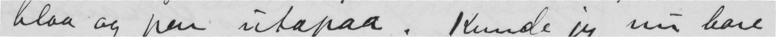blaa og pen utapaa. Kunde jeg nu bare
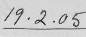19.2.05
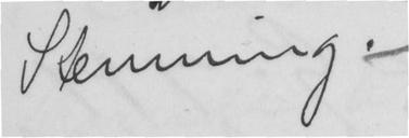Stemning.
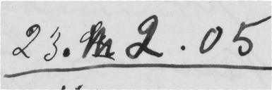23. 2.05
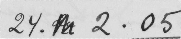24. 2.05
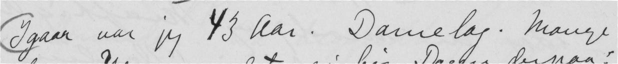Igaar var jeg 43 aar. Damelag. Mange
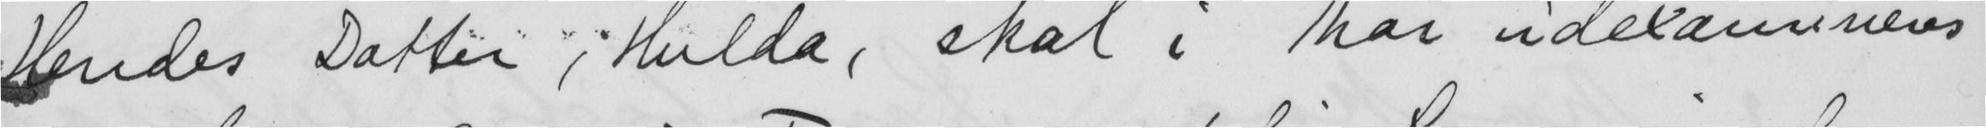Hendes Datter; Hulda, skal i Mai udexamineres
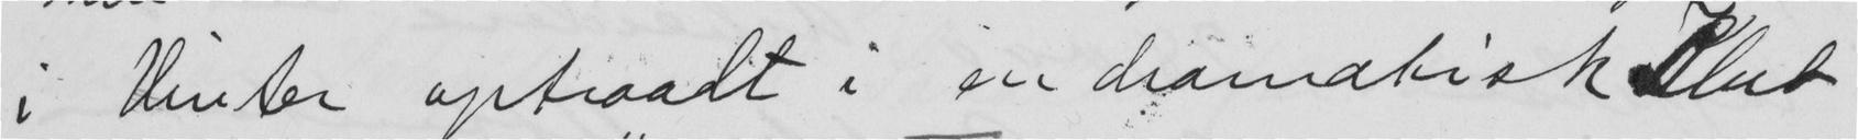i Vinter optraadt i en dramatisk Klub
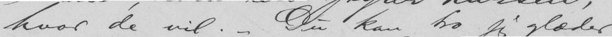hvor de vil. - Du kan tro jeg glæder
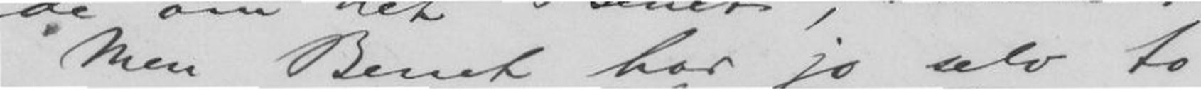jeg. Men Benet har jo selv to
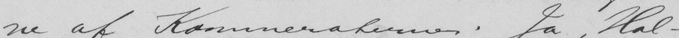ne af Kammeraterne. Ja, Hal-
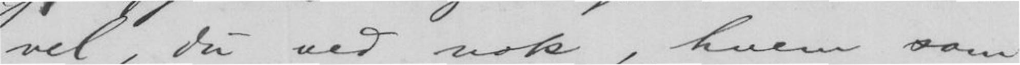vel, du ved nok, hvem som
